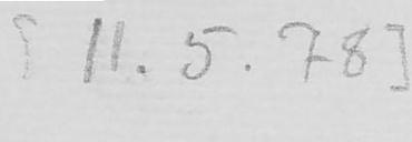[11.5. 78]
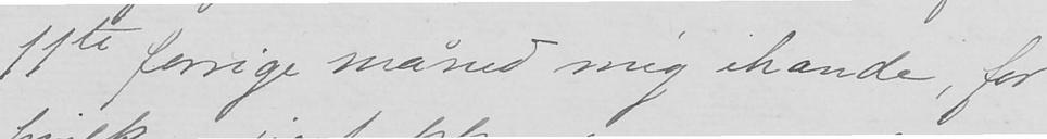11te forrige måned mig ihænde, for
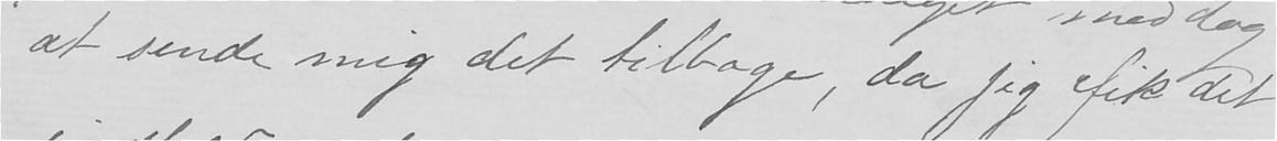at sende mig det tilbage, da jeg fik det
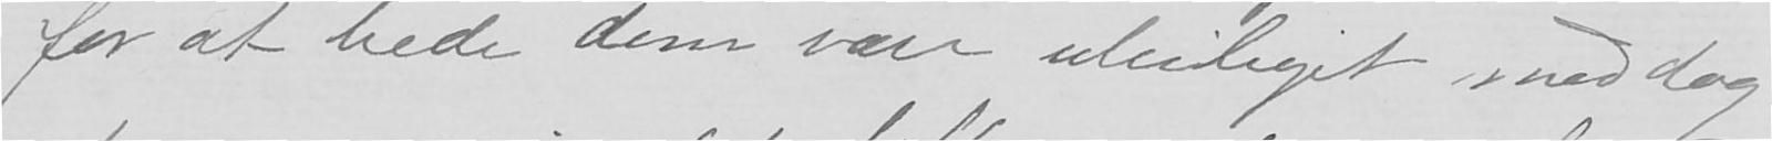for at bede dem være uleiliget med dog
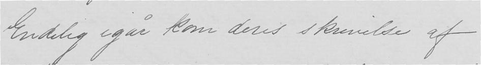Endelig igår kom deres skrivelse af
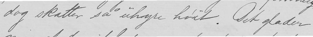dog skatter sa uhyre høit. Dit glæder
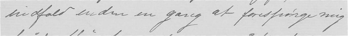indfald endnu en gang at forespørge mig
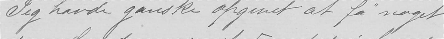Jeg havde ganske opgivet at få noget
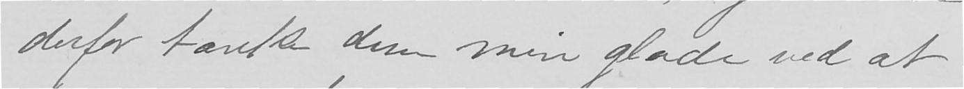derfor tænke dem min glæde ved at
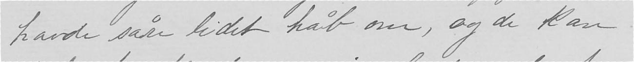havde såre lidet håb om, og de kan
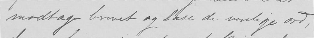modtage brevet og læse de venlige ord,
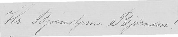Hr. Bjørnstjerne  Bjørnson!
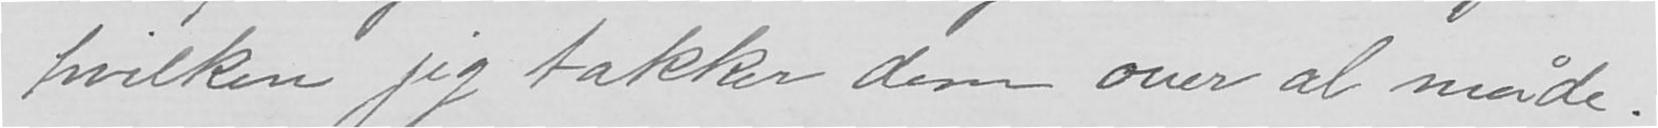hvilken jeg takker dem over al måde.
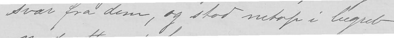svar fra dem, og stod netop i begreb
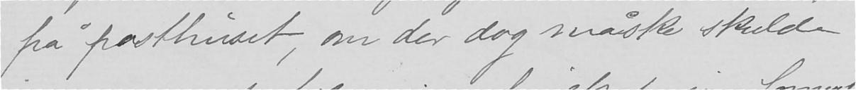på posthuset, om der dog måske skulde
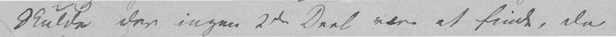Skulde der ingen 2den Deel være at finde, da
